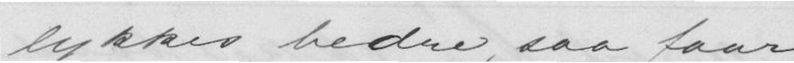lykkes bedre, saa faar
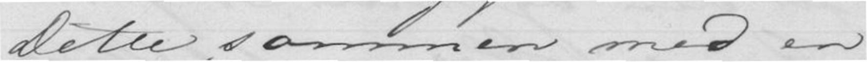Dette, sammen med en
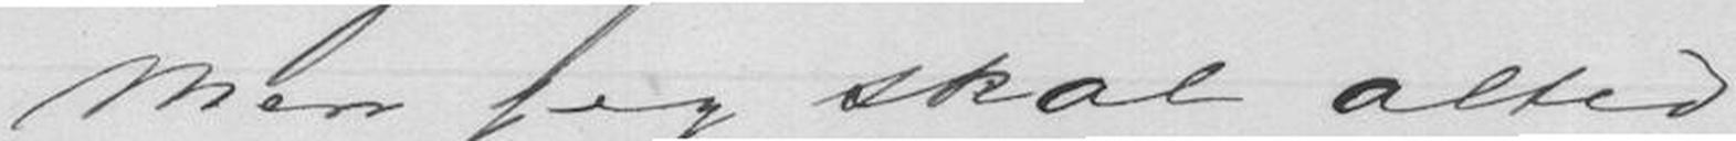Men jeg skal altid
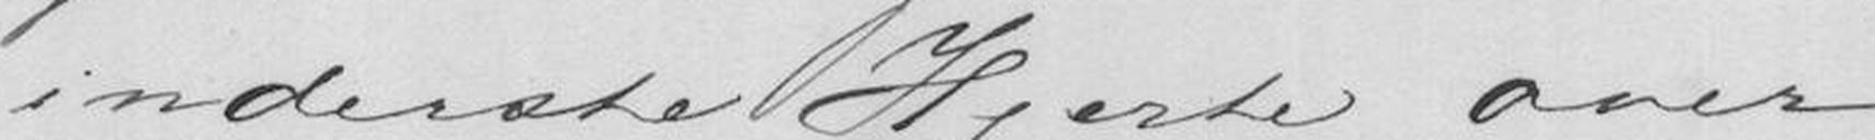inderste Hjerte over
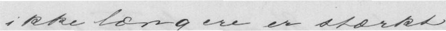ikke længere er stærkt
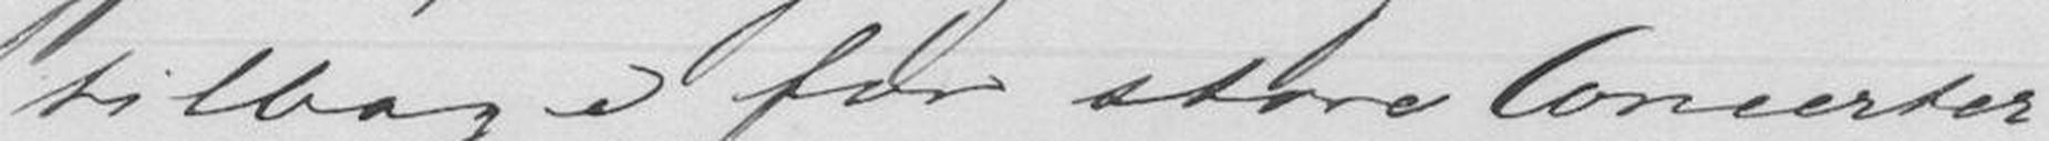tilbage for store Concerter.
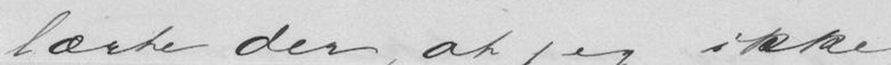lærte der, at jeg ikke
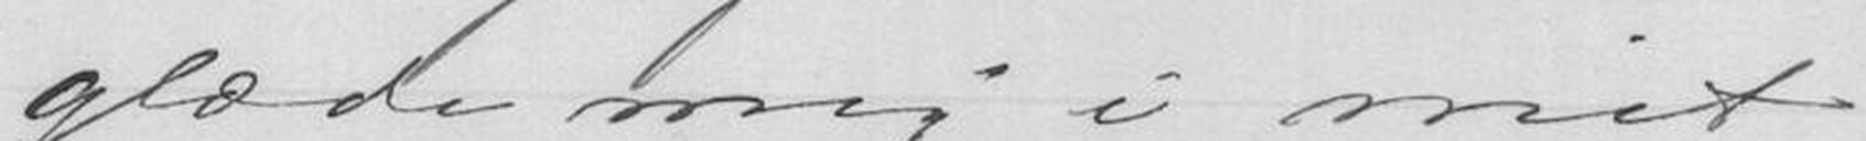glæde mig i mit
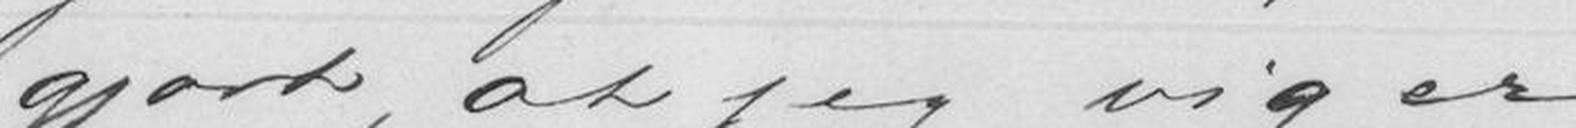gjort, at jeg viger
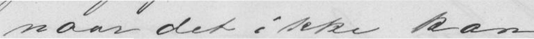naar det ikke kan
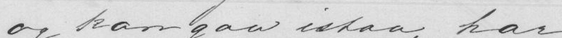og kan gaa istaa, har
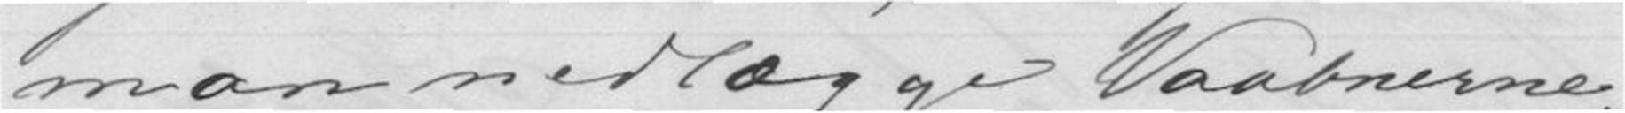man nedlægge Vaabnerne.
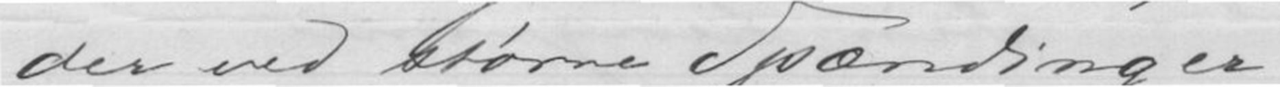der ved større Spændinger
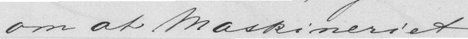om at Maskineriet
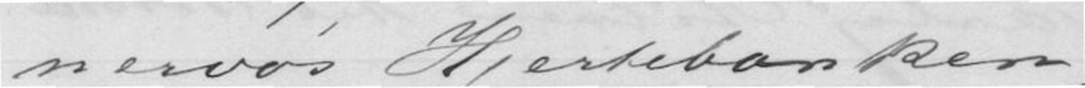nervøs Hjertebanken,
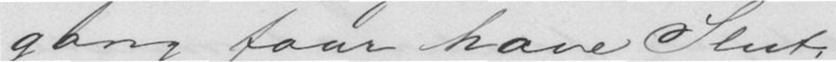gang faar have Slut;
